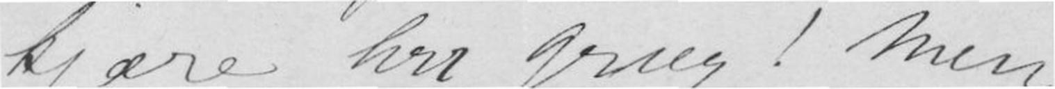kjære hrr Grieg! Men
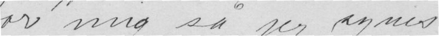for mig så jeg synes
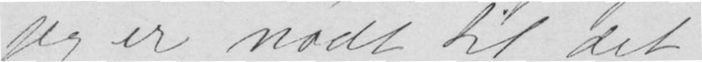jeg er nødt til det!
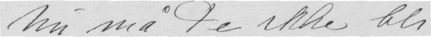Nu må De ikke bli
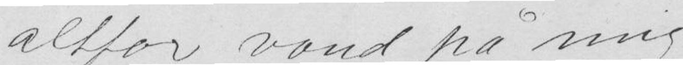altfor vond på mig
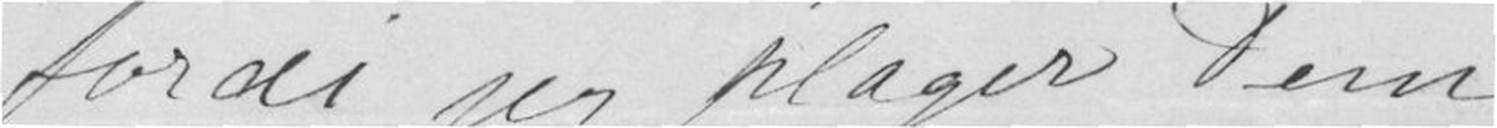fordi jeg plager Dem
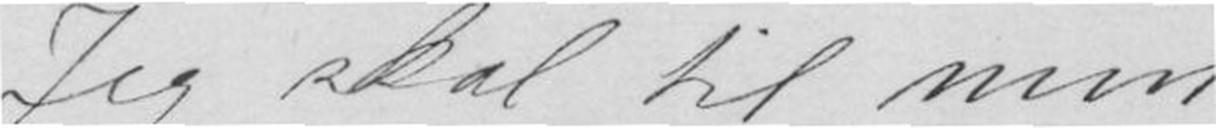Jeg skal til min
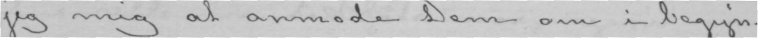jeg mig at anmode Dem om i begyn-
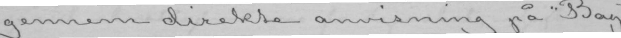gennem direkte anvisning på «Bay-
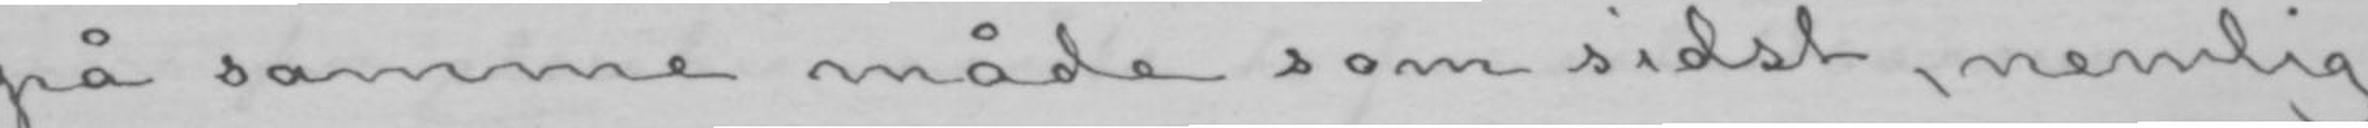på samme måde som sidst, nemlig
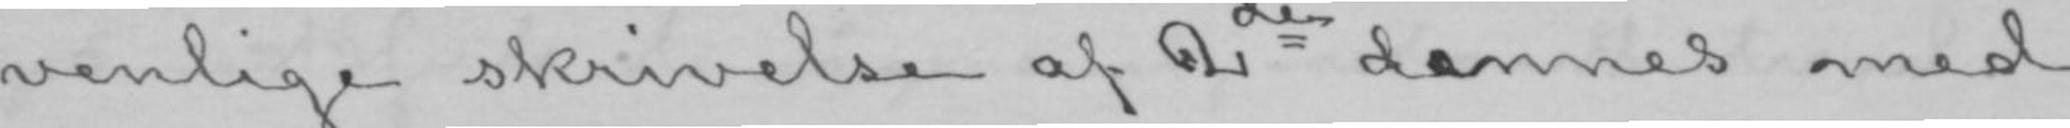venlige skrivelse af 2den dennes med
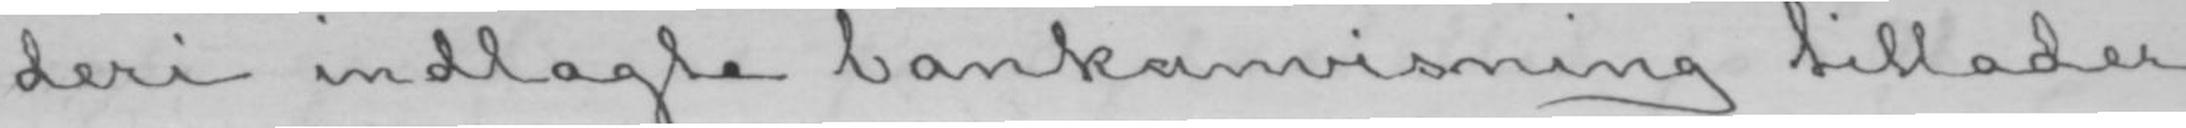deri indlagte bankanvisning tillader
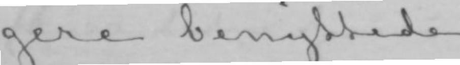gere benyttede.
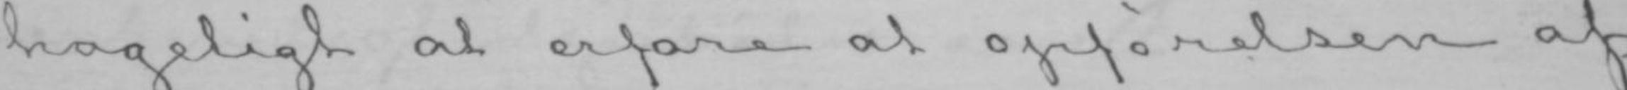hageligt at erfare at opførelsen af
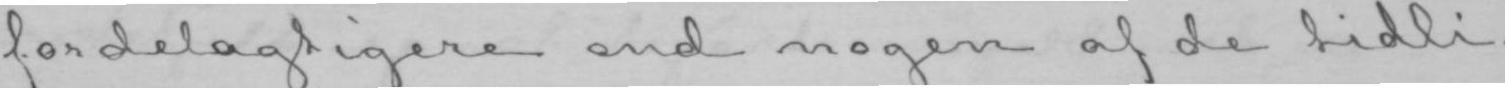fordelagtigere end nogen af de tidli-
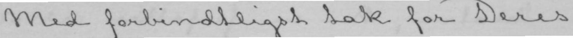Med forbindtligst tak for Deres
